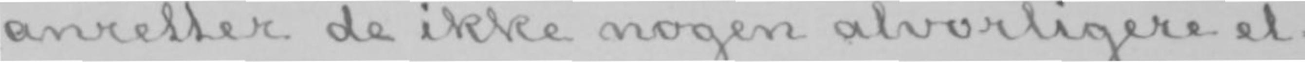anretter de ikke nogen alvorligere el-
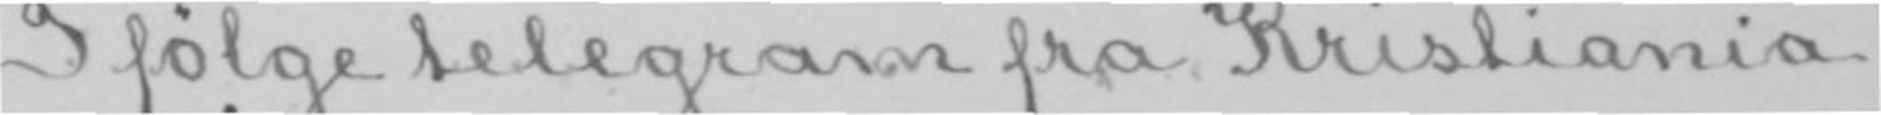Ifølge telegram fra Kristiania
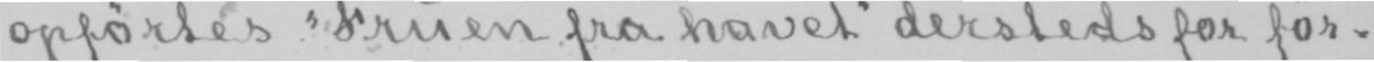opførtes «Fruen fra havet» dersteds for før-
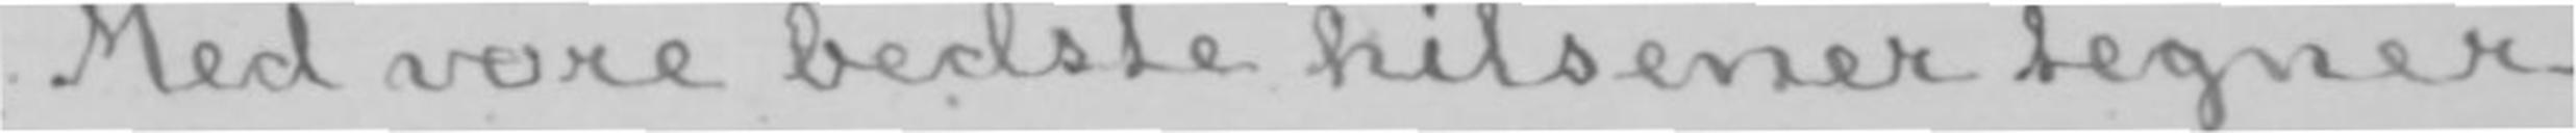Med vore bedste hilsener tegner
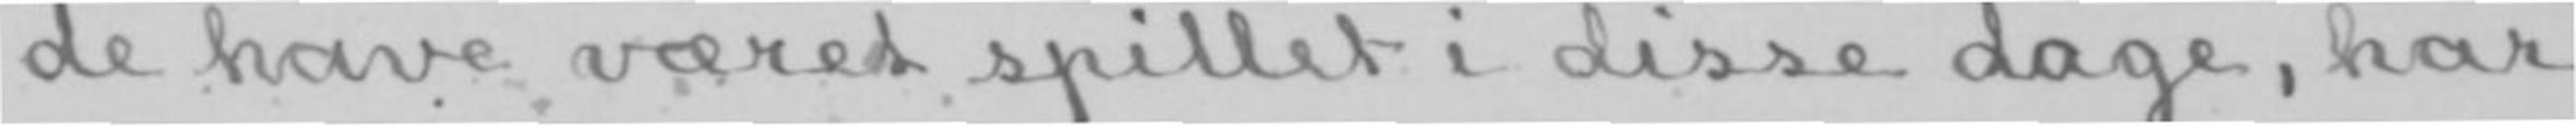de have været spillet i disse dage, har
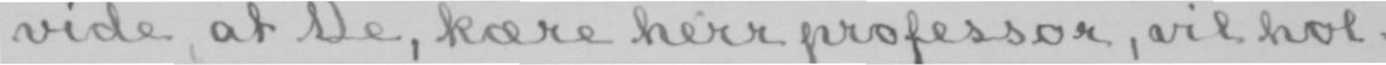vide at De, kære herr professor, vil hol-
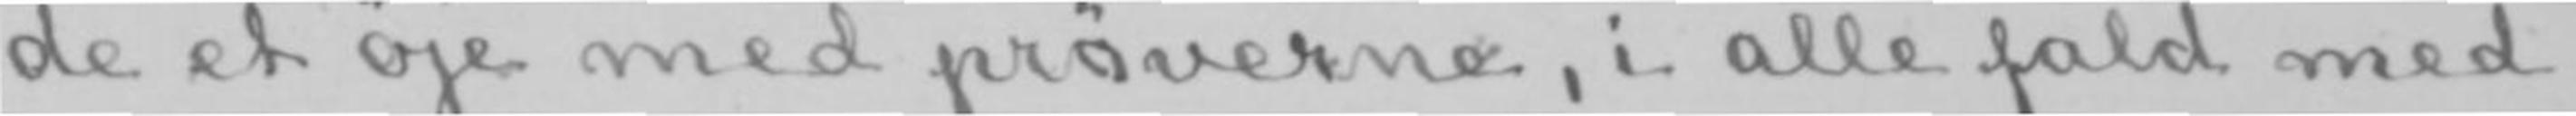de et øje med prøverne, i alle fald med
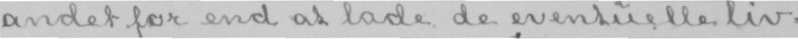andet for end at lade de eventuelle liv-
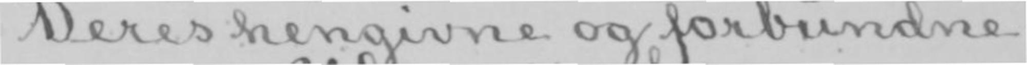Deres hengivne og forbundne
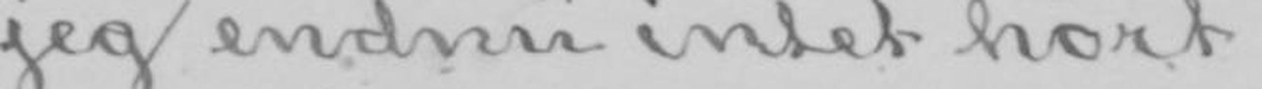jeg endnu intet hørt.
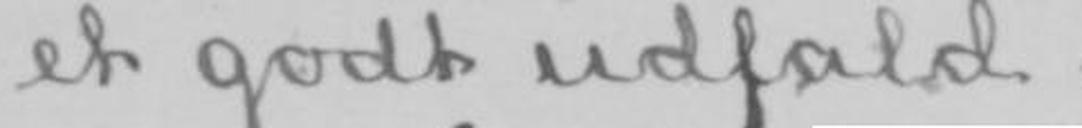et godt udfald.
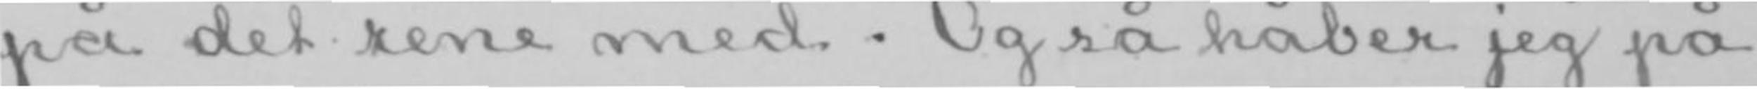på det rene med. Og så håber jeg på
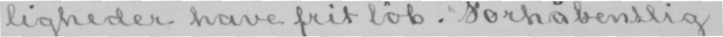ligheder have frit løb. Forhåbentlig
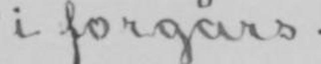i forgårs.
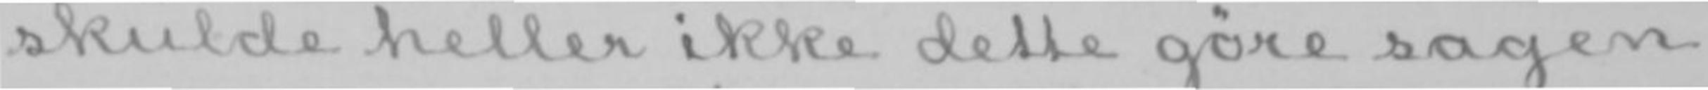skulde heller ikke dette gøre sagen
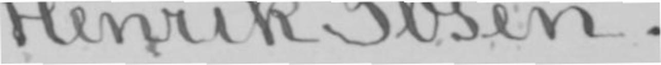Henrik Ibsen.
